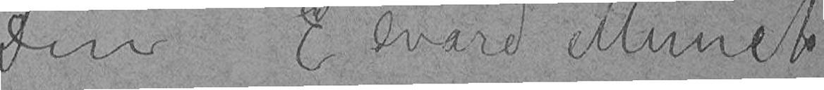Din Edvard Munch
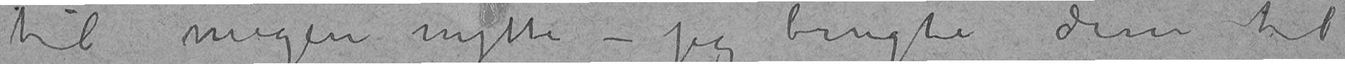til megen nytte – jeg brugte dem til
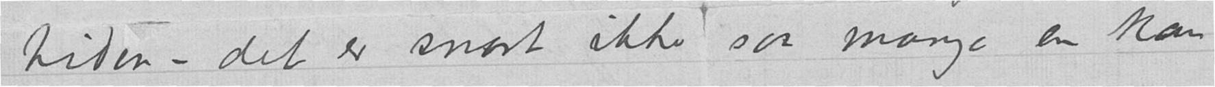tiden – det er snart ikke saa mange en kan
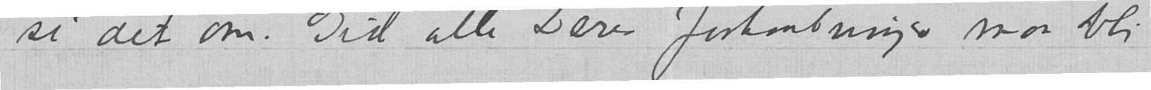si det om. Gid alle Deres forhaabninger maa bli
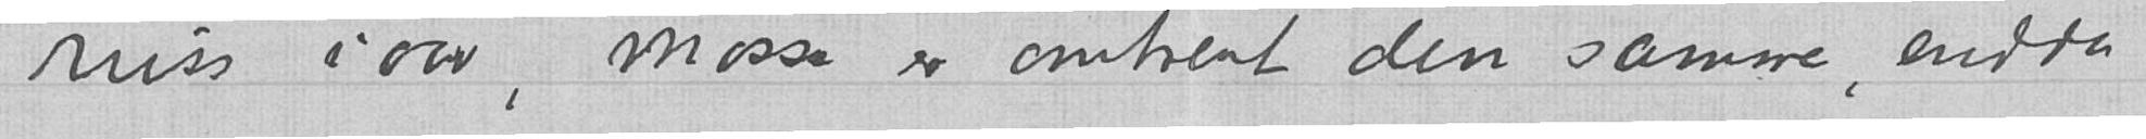russ iaar, Mosse er omtrent den samme, endda
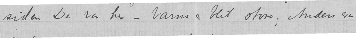siden De var her – barna er blit store; Anders er
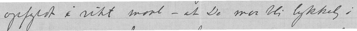opfyldt i rikt maal – at De maa bli lykkelig i
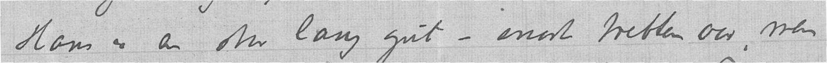Hans er en stor lang gut – snart tretten aar, men
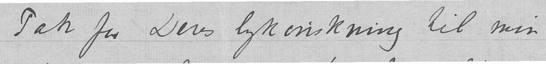Tak for Ders lykønskning til min
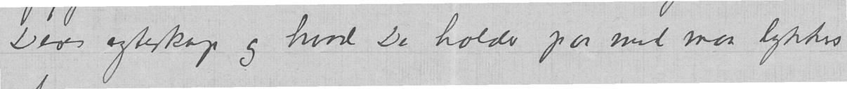Deres ægteskap og hvad De holder paa med maa lykkes
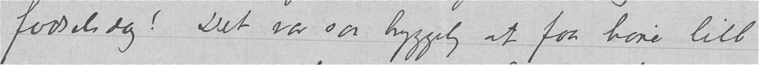fødselsdag! Det var saa hyggelig at faa høre litt
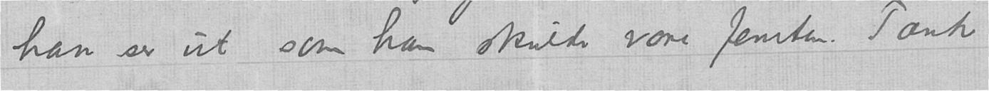han ser ut som han skulde være femten. Tænk
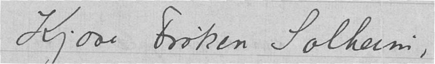Kjære Frøken Solheim,
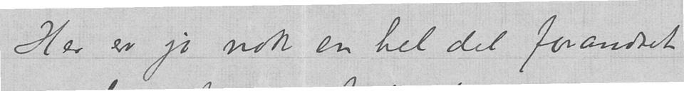Her er jo nok en hel del forandret
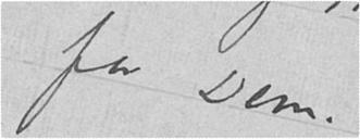for Dem.
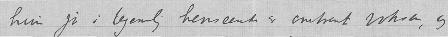hun jo i legemlig henseende er omtrent voksen, og
